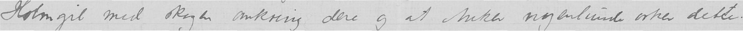med skogen omkring dere og at Anker nogenlunde orker dette.
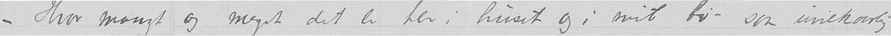Hvor mangt og meget det er her i huset og i mit liv- som uvilkaarlig
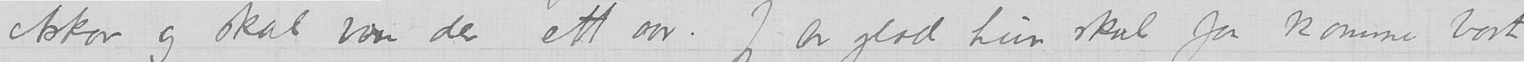Askov og skal være der ett aar. Jeg er glad hun skal faa komme bort
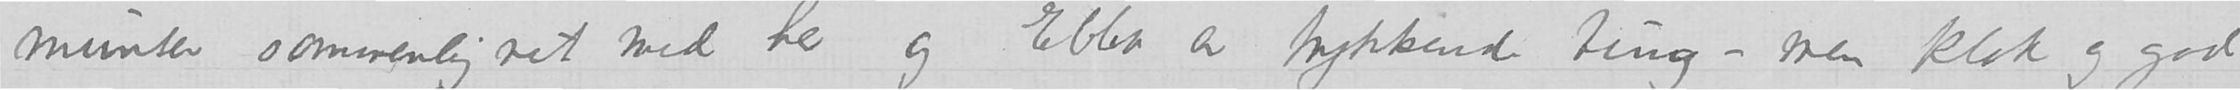munter sammenlignet med her og Ebba er trykkende tung - men klok og god
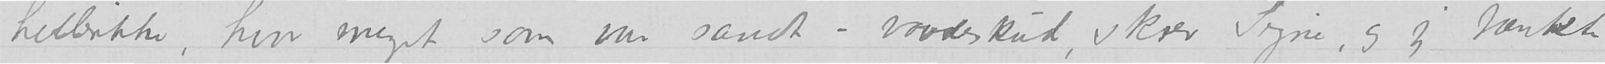hellerikke, hvor meget som var sandt- vaadeskud, skrev Signe, og jeg tænkte
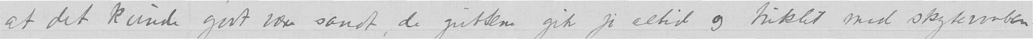at det kunde godt være sandt, de guttene gik jo altid og tuklet med skytevaaben.
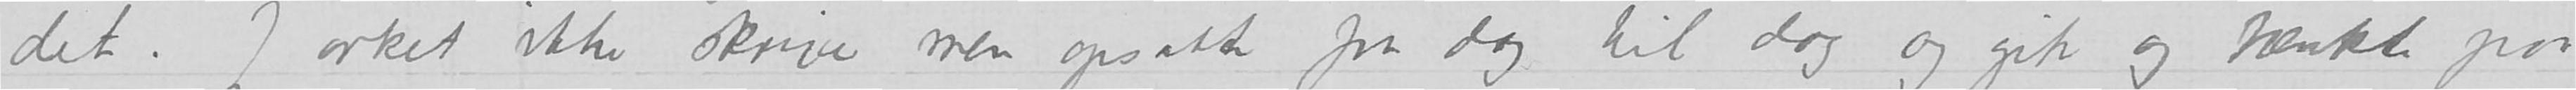det. Jeg orket ikke skrive men opsatte fra dag til dag og gik og tænkte paa
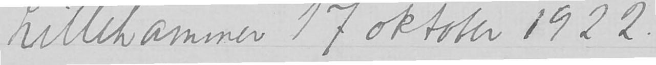Lillehammer 17. oktober 1922
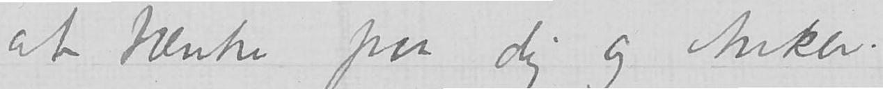tænke paa dig og Anker.
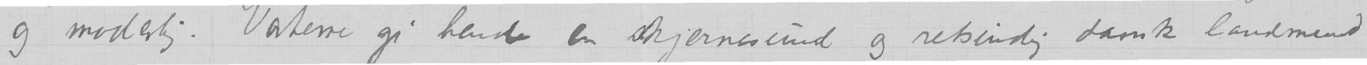og moderlig. Vorherre gi hende en kjernesund og retsindig dansk landmand
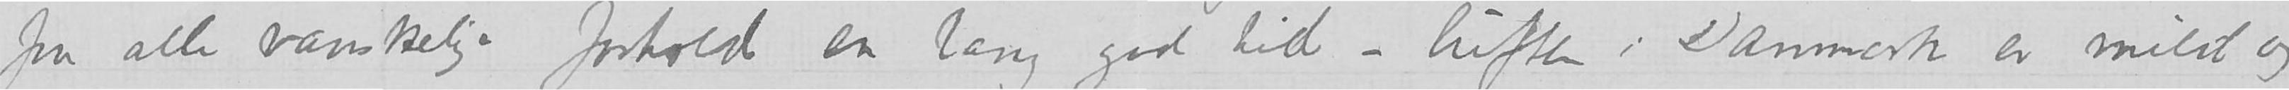fra alle vanskelige forhold en lang god tid. luften i Danmark er mild og
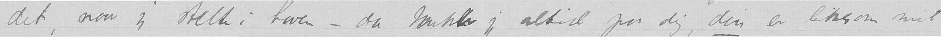naar jeg stelte i haven - da tænkte jeg altid paa dig, din er likesom mit
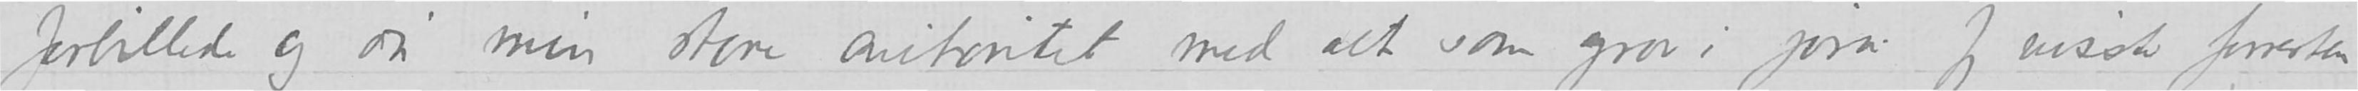forbillede og du min store autoritet med alt som gror i jora. Jeg visste forresten
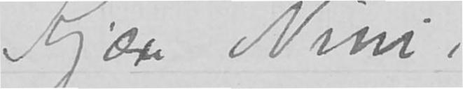Kjære Nini
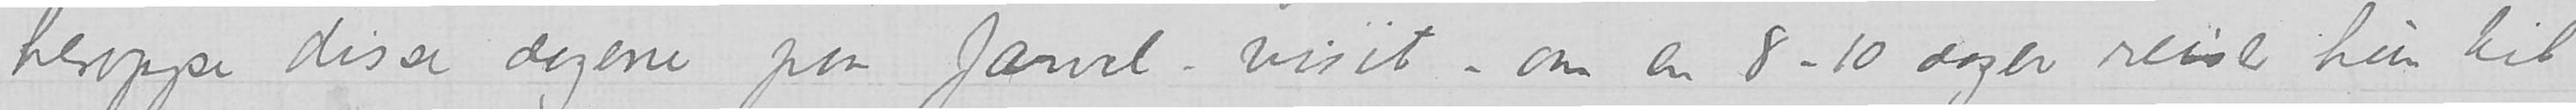heroppe disse dagene paa farvel-visit. om en 8-10 dager reiser hun til
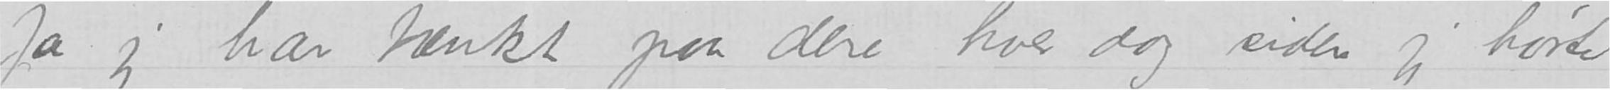Ja jeg har tænkt paa dere hver dag siden jeg hørte
